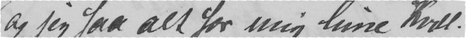og jeg saa alt for mig lune Kvæl-
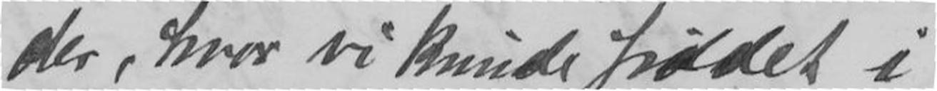der, hvor vi kunde siddet i
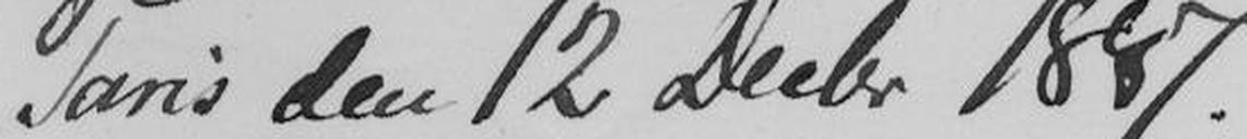Paris den 12 Decbr 1887.
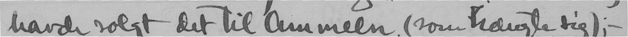havde solgt det til Ammeln, (som hængte sig); -
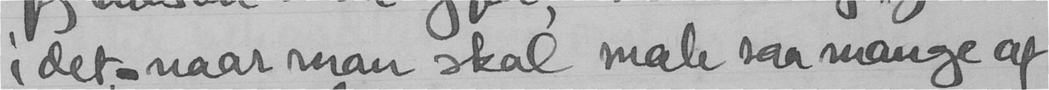i det, - naar man skal male saa mange af
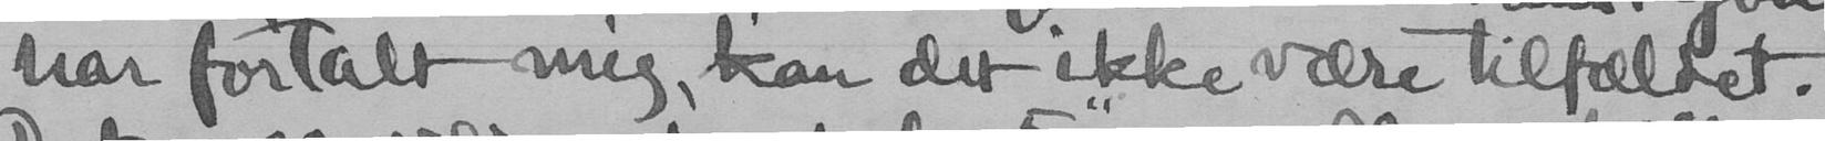har fortalt mig, kan det ikke være tilfældet.
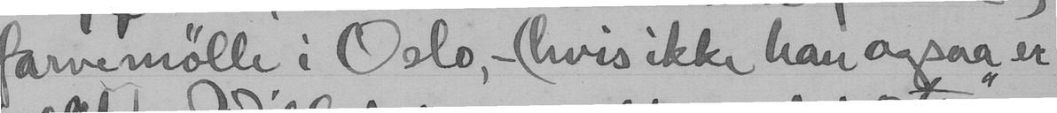farvemølle i Oslo,- (hvis ikke han ogsaa er
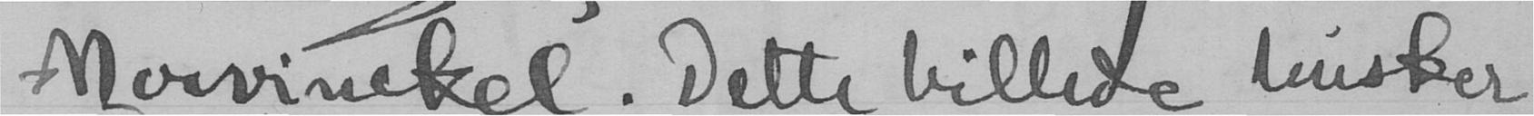Mowinckel. Dette billede husker
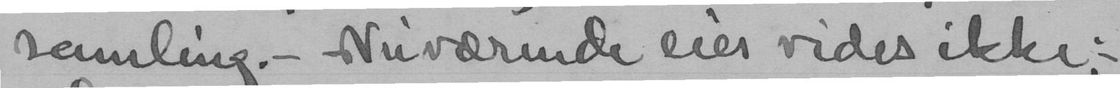samling.- Nuværende eier vides ikke; -
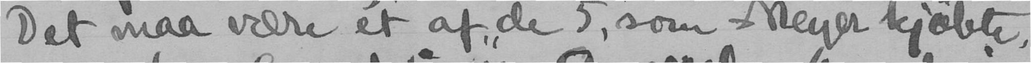Det maa være et af "de 5, som Meyer kjøbte,
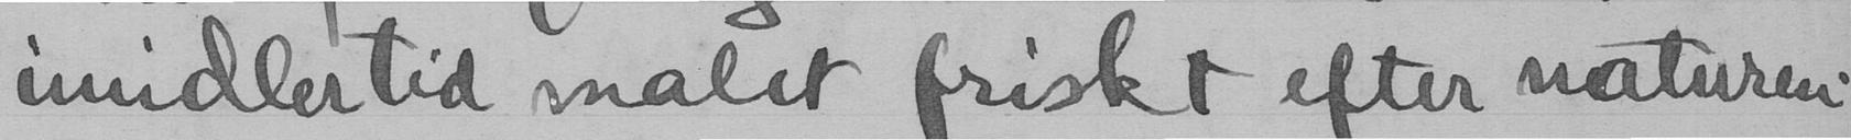imidlertid malet friskt efter naturen
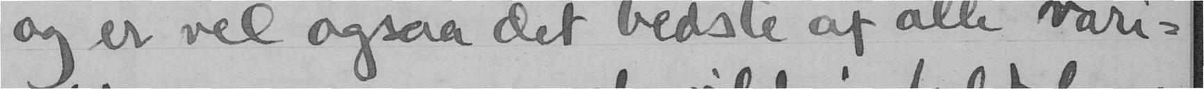og er vel ogsaa det bedste af alle vari-
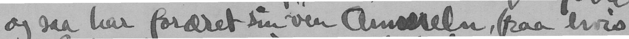og saa har foræret sin ven Ammeln, (paa hvis
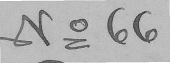No 66
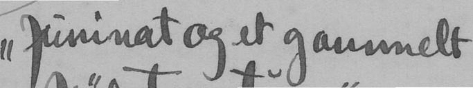"Juninat og et gammell
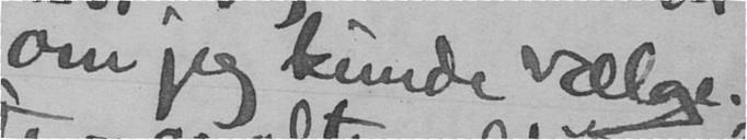om jeg kunde vælge.
